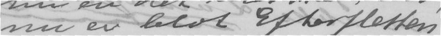nu er blot Eftersletten
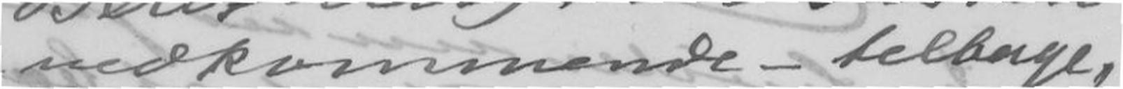vedkommende - tilbage,
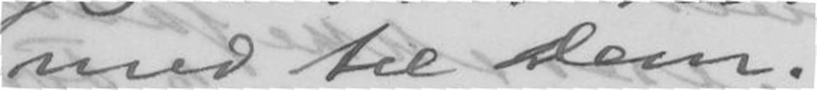med til Dem.
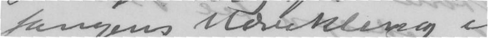sangens Udvikling i
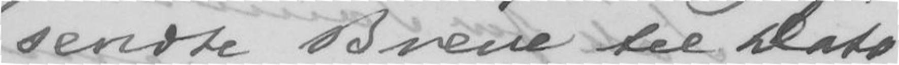sendte Breve til Dato
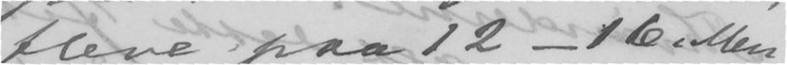flere paa 12 - 16. Men
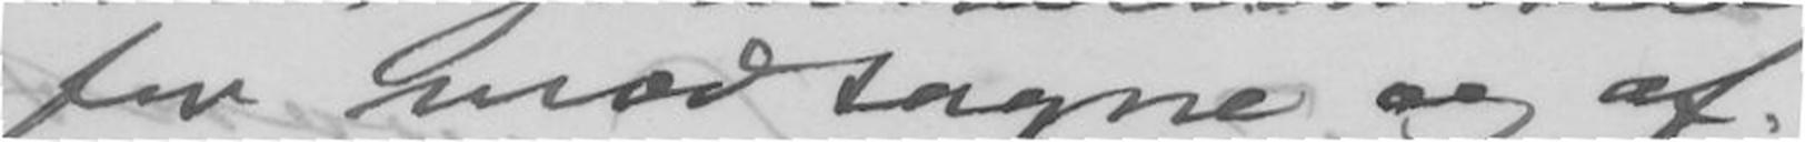for modtagne og af-
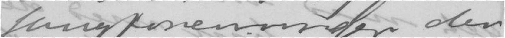sangforreninger der
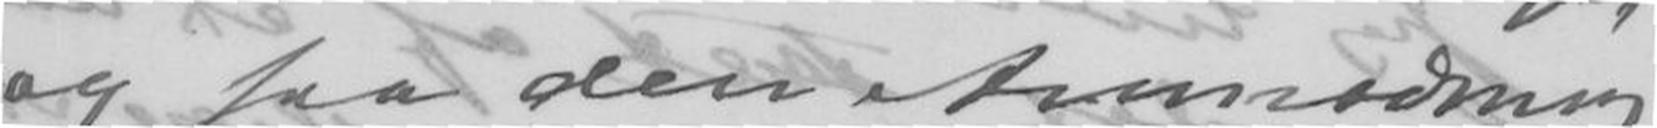og saa den Anmodning
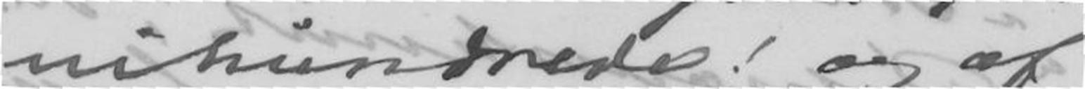- nihundrede! og af
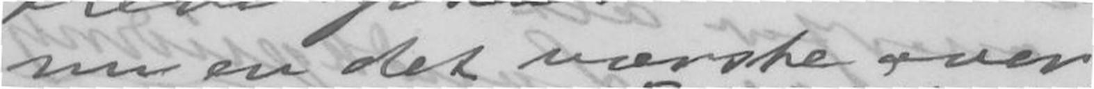nu er det værste over,
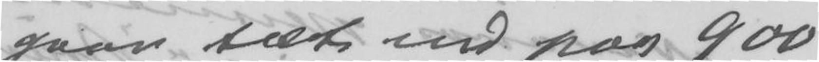gaar tæt ind paa 900
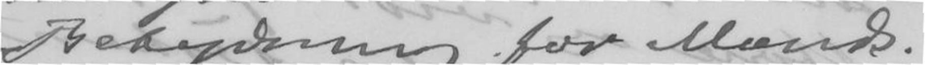Betydning for Mands-
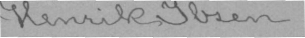Henrik Ibsen.
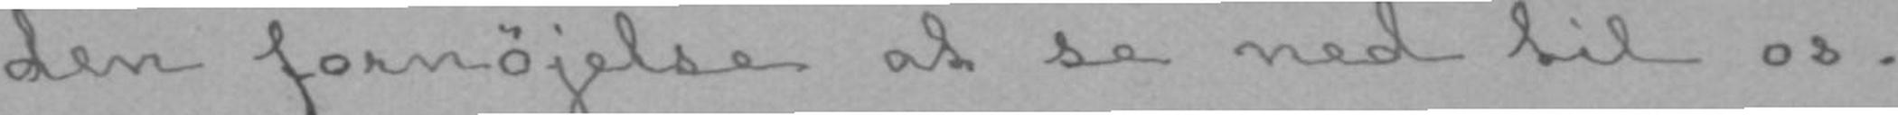den fornøjelse at se ned til os.
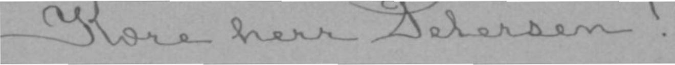Kære herr Petersen!
